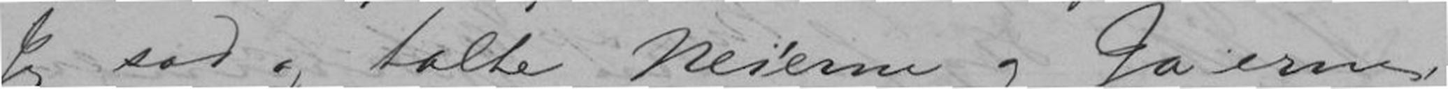Jeg sad og talte Nei'erne og Ja'erne,
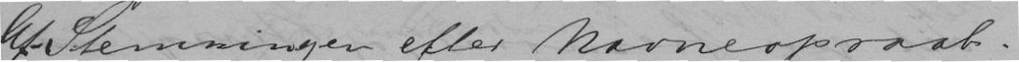Af-Stemningen efter Navneopraab.
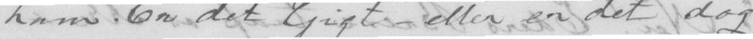ham. Er det Gigt - eller er det dog
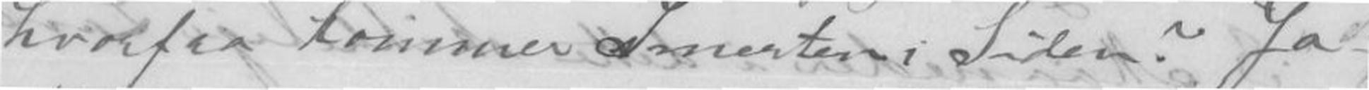hvorfra kommer Smerten i Siden? Ja
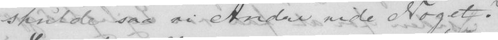skulde saa vi Andre vide Noget?
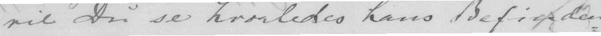vil Du se hvorledes hans Befinden-
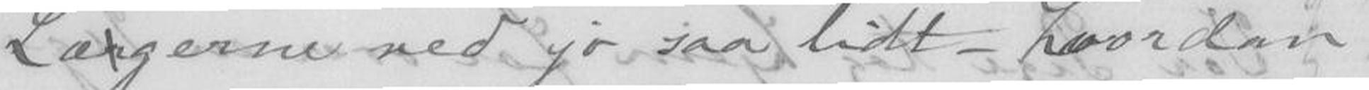Lægerne ved jo saa lidt - hvordan
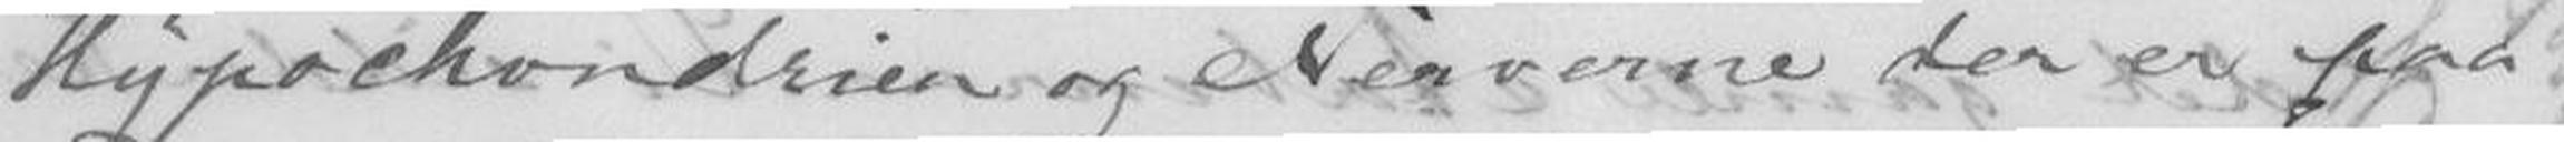Hypochondrien og Nerverne der er paa
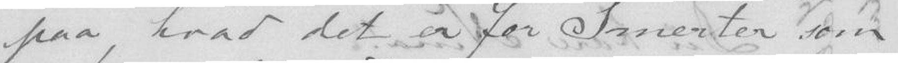paa, hvad det er for Smerter som
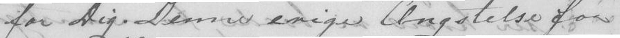for Dig. Denne evige Ængstelse for
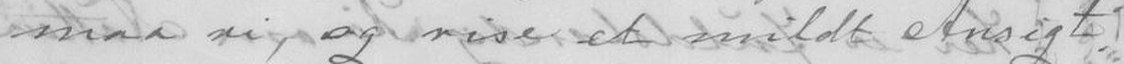maa vi, og vise et mildt Ansigt.
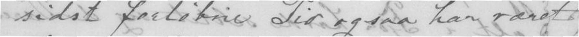sidst forløbne Tid ogsaa har været
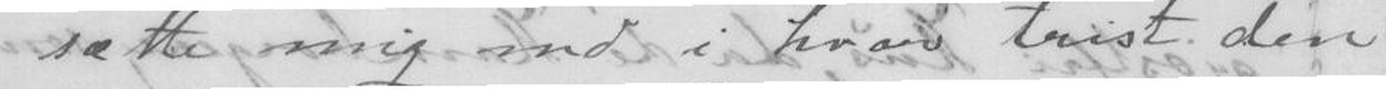sætte mig ind i hvor trist den
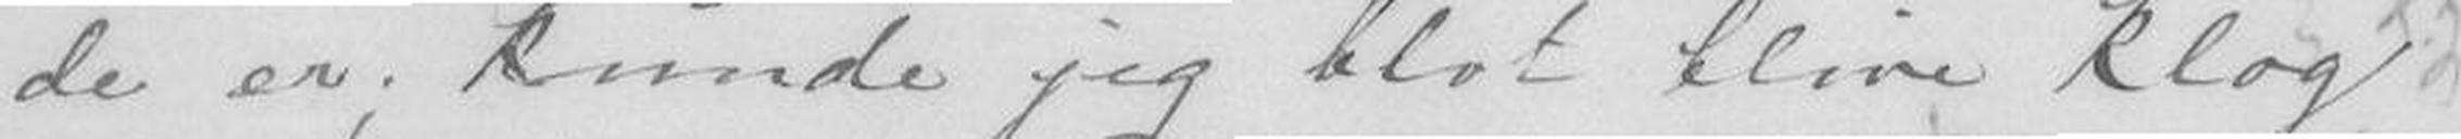de er; kunde jeg blot blive klog
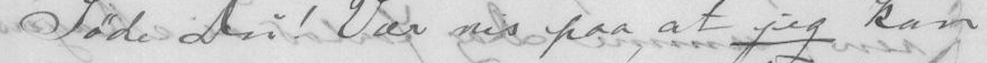Søde Du! Vær vis paa at jeg kan
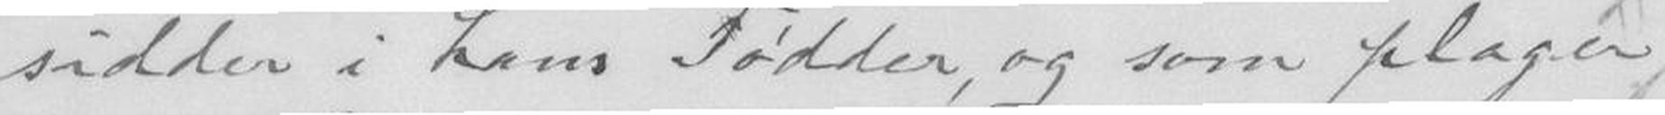sidder i hans Fødder, og som plager
